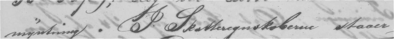myntning. P. Skatteregnskaberne staaer
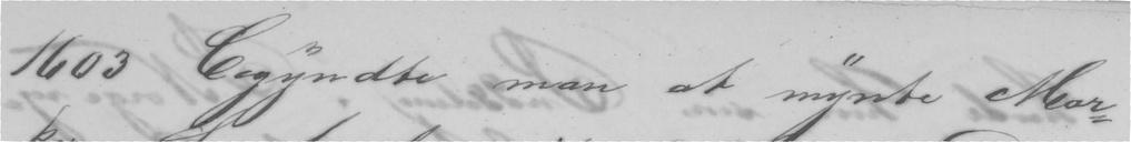1603 begyndte man at mynte Mar-
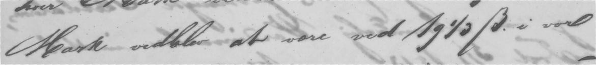Mark vedblev at være ved 19⅓ ẞ. i vor
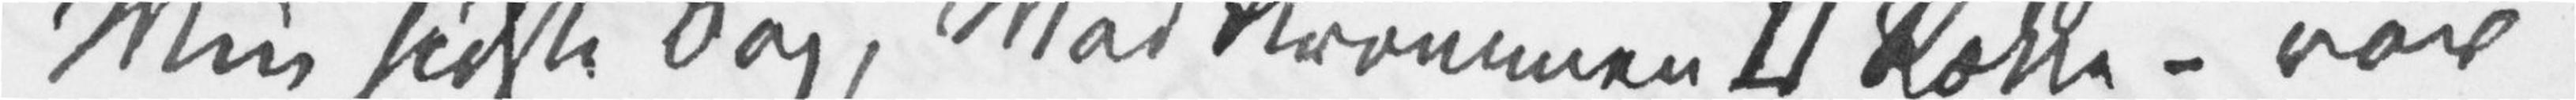Min sidste Bog, «Mod Strømmen II Række» – var
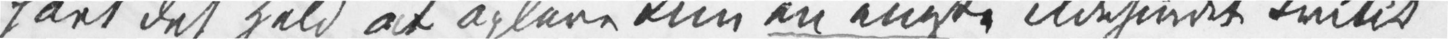havt det Held at opleve kun en eneste ildesindet Kritik
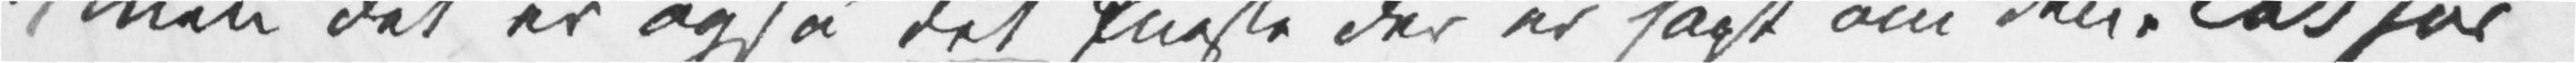men det er også det Eneste der er sagt om den. Tak for
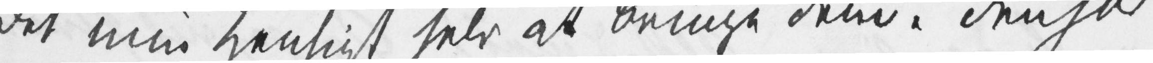det min Hensigt selv at bringe Dem. Den har
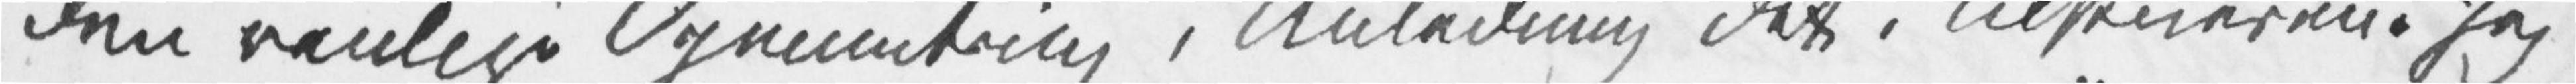den venlige Opmuntring i Anledning det i Tilskueren. Jeg
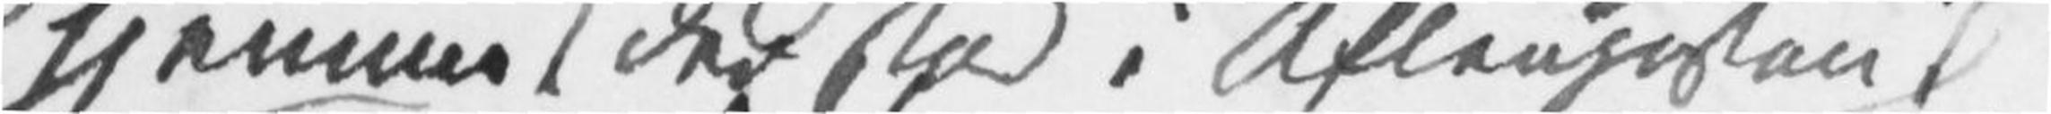hjemme (den stod i «Aftenposten»),
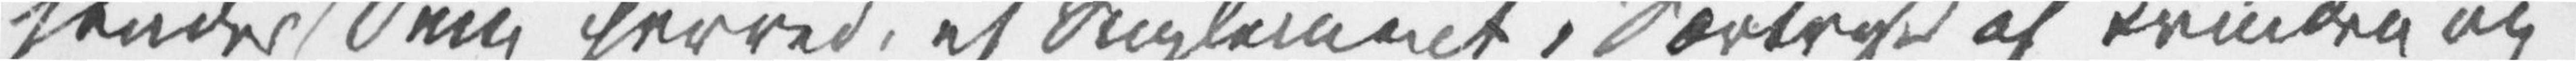sender Dem herved, et Suplement, Særtryk af «Kvinden og
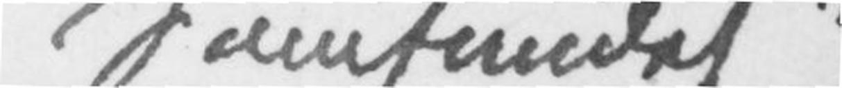Samfundet»[.]
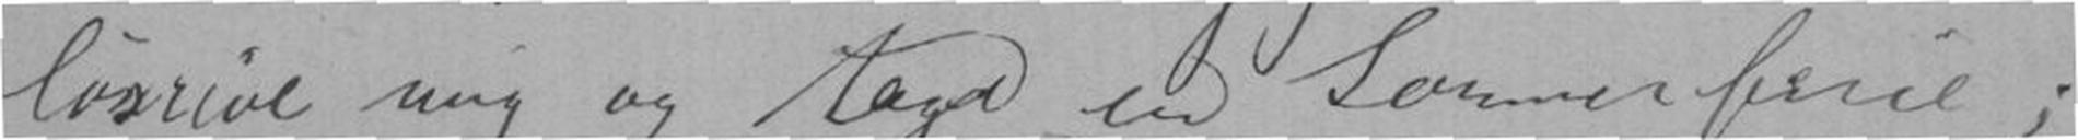løsrive mig og tage en Sommerferie;
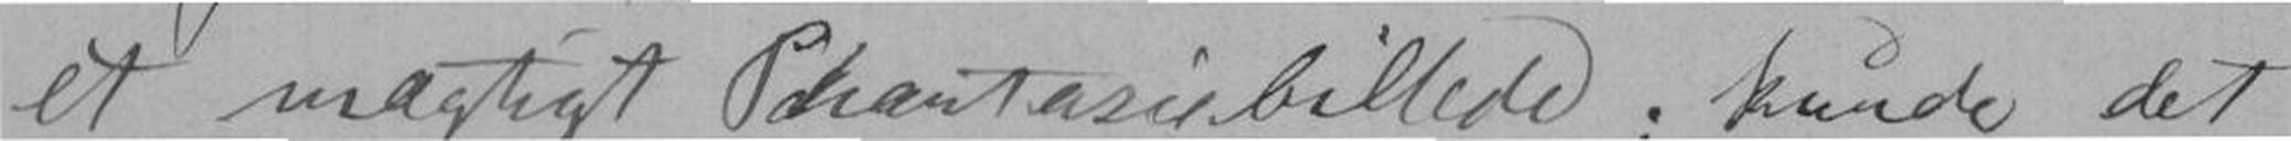et mægtigt Phantasiebillede; kunde det
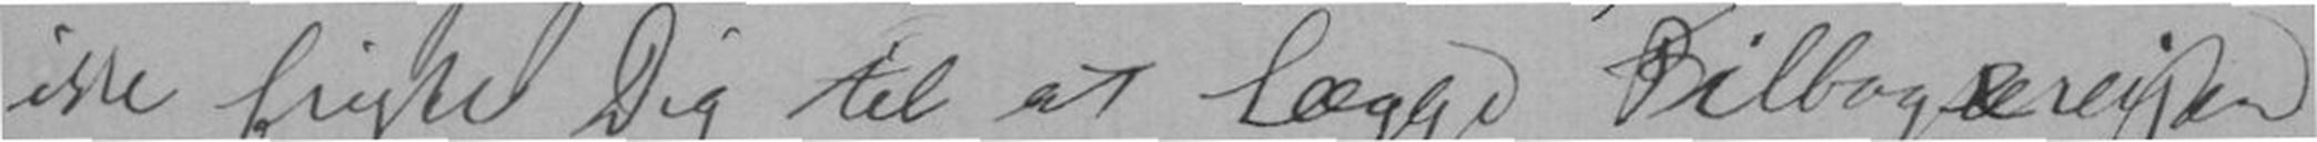ikke fristet Dig til at lægge Tilbagereisen
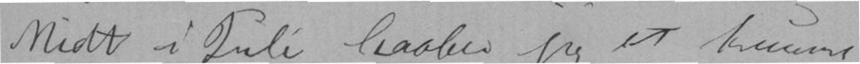Midt i Juli haaber jeg at kunne
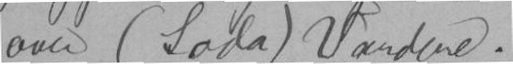over (Soda) Vandene.
